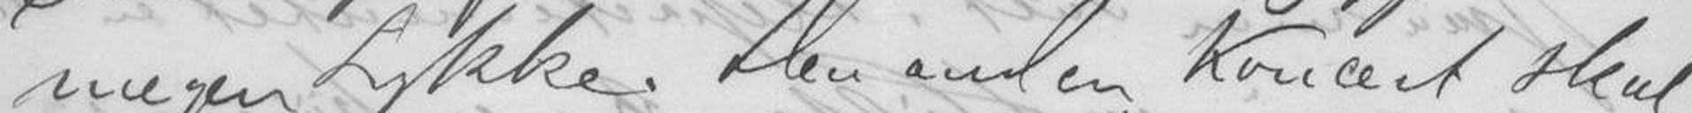megen Lykke. Den anden Koncert skal
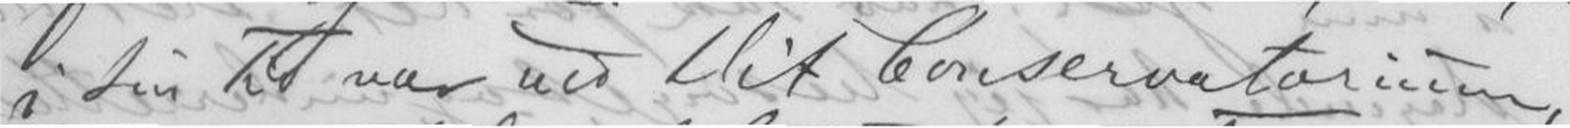i sin Tid var ved Dit Conservatorium,
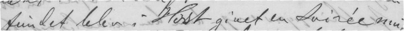fundet blev i Høst givet en Soirée mu-
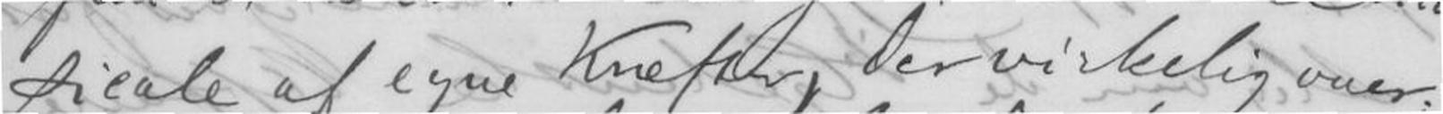sicale af egne Krefter, der vi virkelig over-
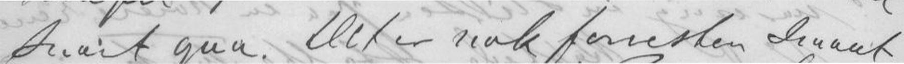snart gaa. Det er nok forresten smaat
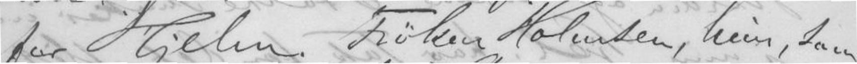for Hjelm. Frøken Holmsen, hun, som
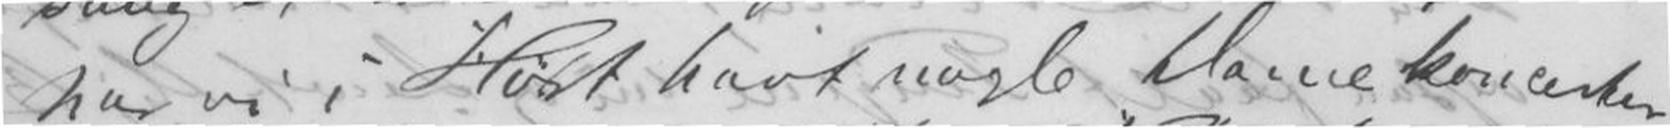har vi i Høst havt nogle Damekoncerter
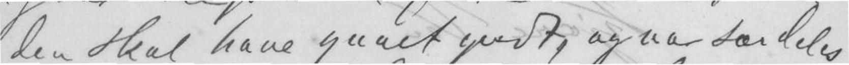den skal have gaaet godt, og var særdeles
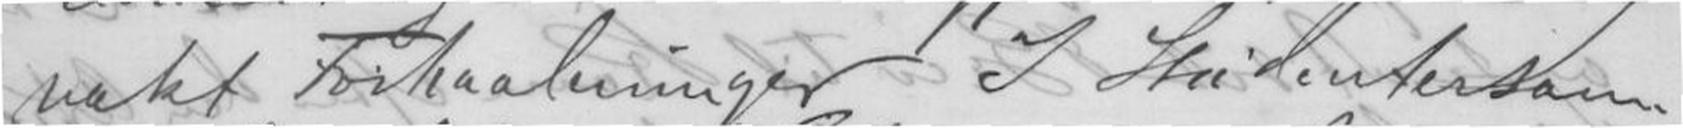vakt Forhaabninger I Studentersam-
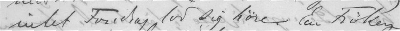intet Foredrag lod sig høre. En Frøken
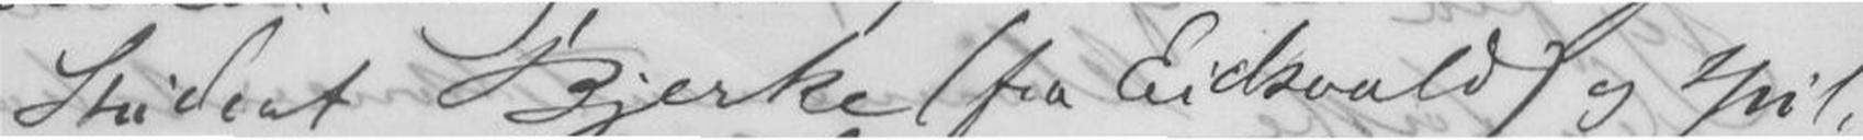Student Bjerke (fra Eidsvold) og spil-
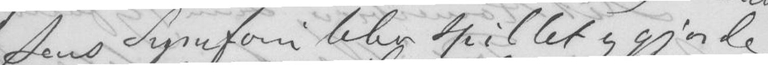sens Symfoni blev spillet og gjorde
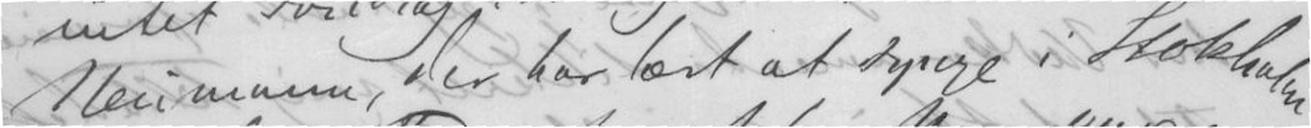Neumann, der har lært at synge i Stockholm
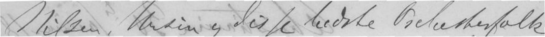Nilsen Ursin og disse bedste Orchesterfolk
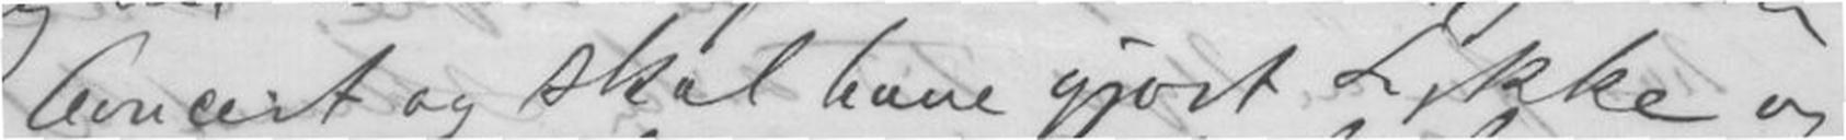Concert og skal have gjort Lykke og
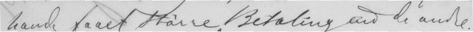havde faaet større Betaling end de andre.
